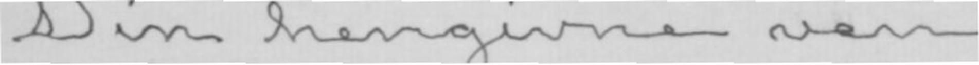Din hengivne ven
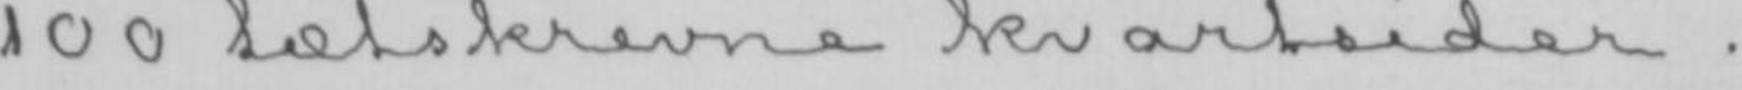100 tætskrevne kvartsider.
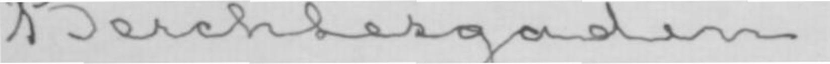Berchtesgaden.
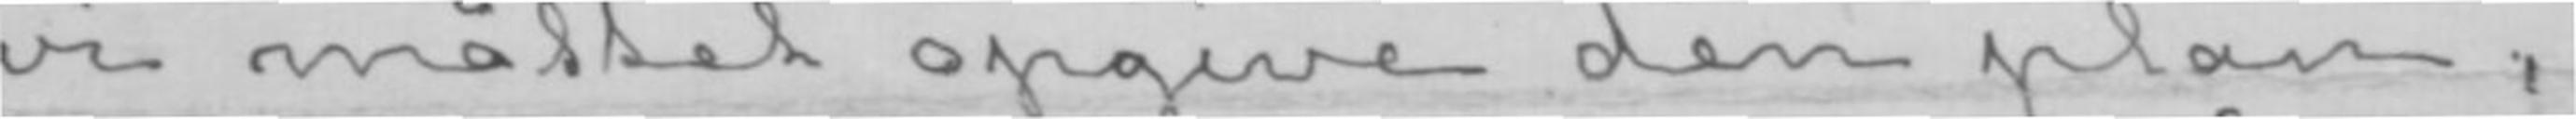vi måttet opgive den plan,
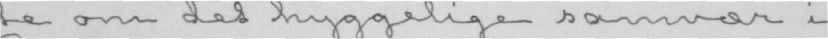te om det hyggelige samvær i
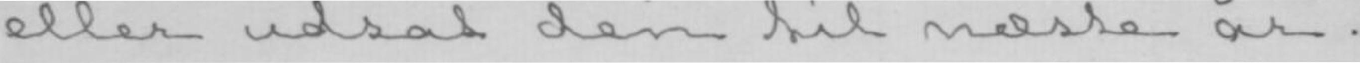eller udsat den til næste år.
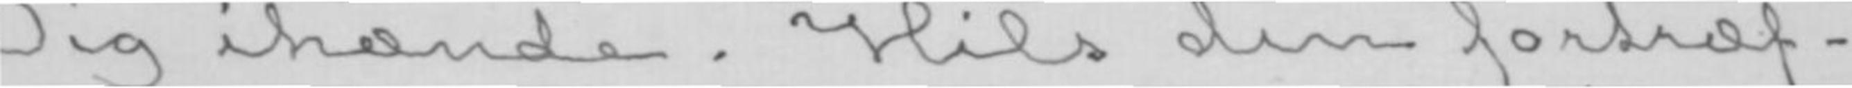Dig ihænde. Hils din fortræf-
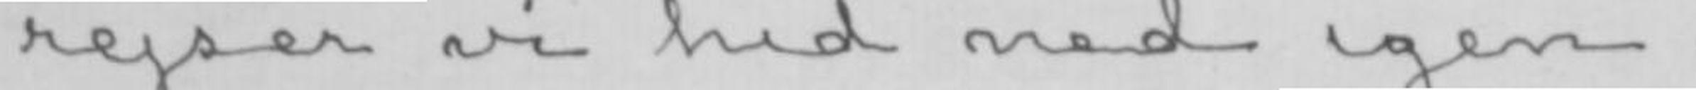rejser vi hid ned igen.
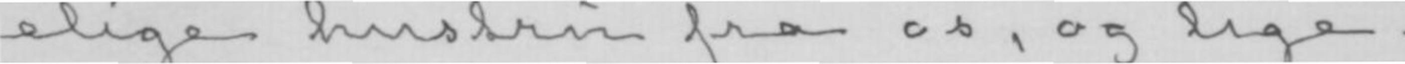felige hustru fra os, og lige-
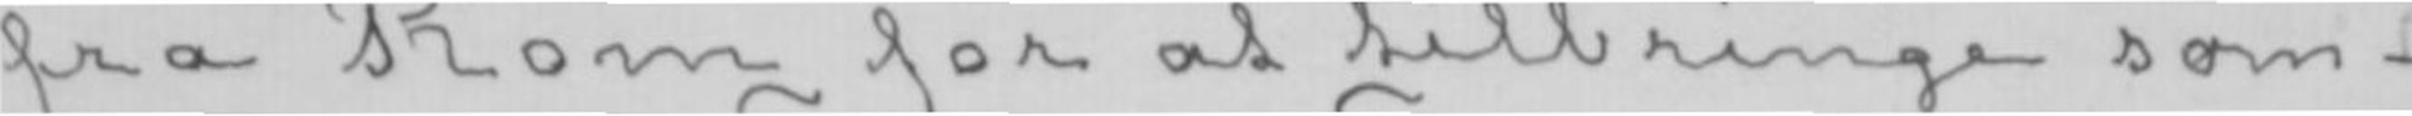fra Rom for at tilbringe som-
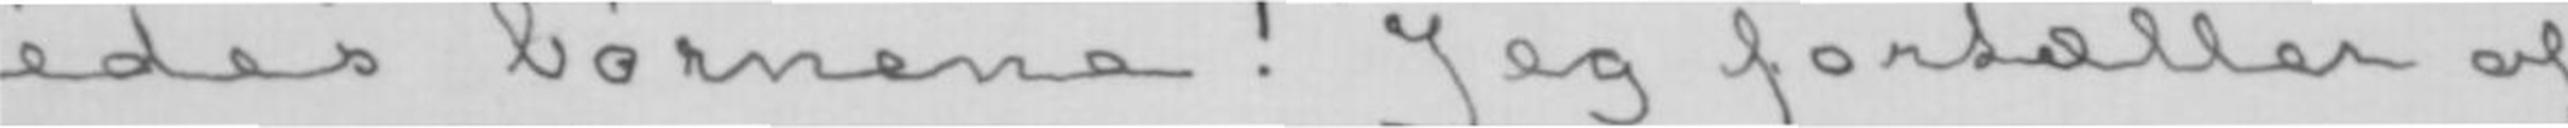ledes børnene! Jeg fortæller of-
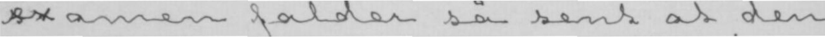sexamen falder så sent at den
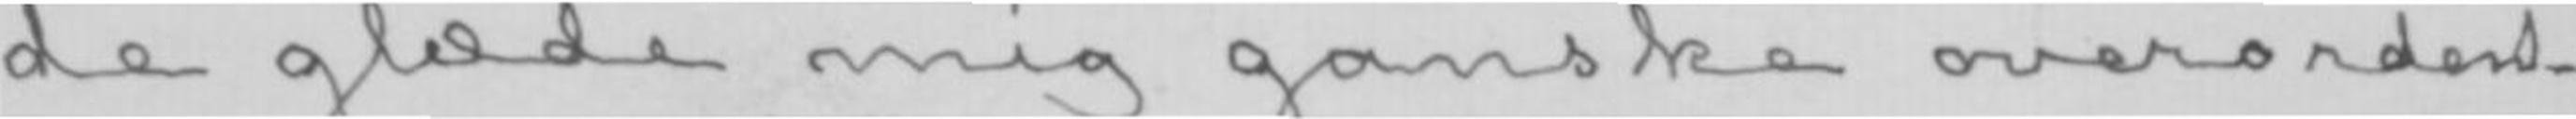de glæde mig ganske overordent-
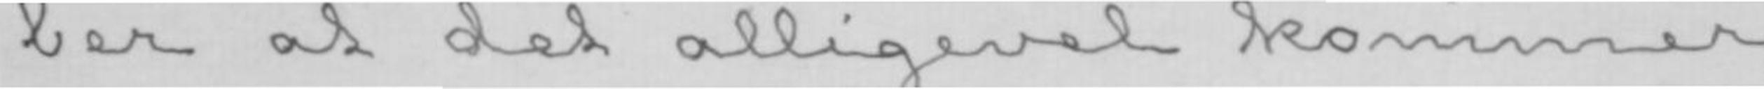ber at det alligevel kommer
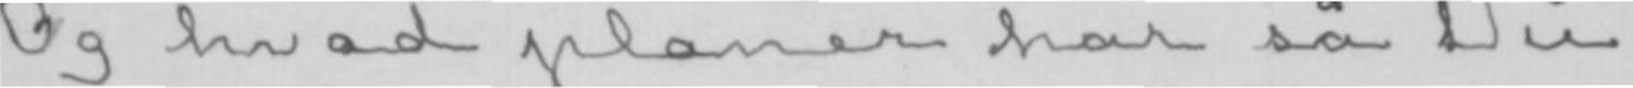Og hvad planer har så Du
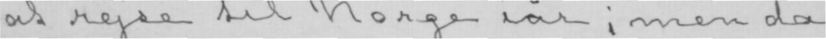at rejse til Norge iår; men da
